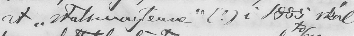at "statsmagterne" (!) i 1885 skal
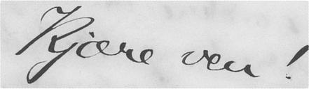Kjære ven!
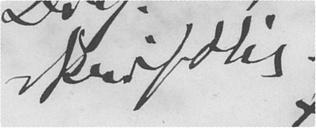skriftlig.
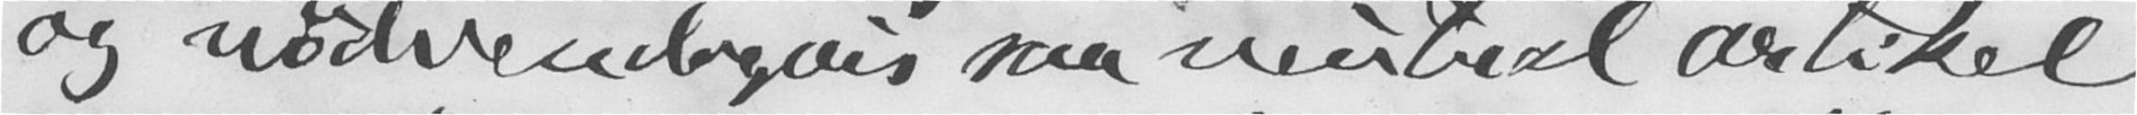og nødvendigvis saa neutral artikel
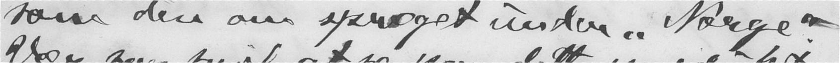som den om sproget under "Norge".
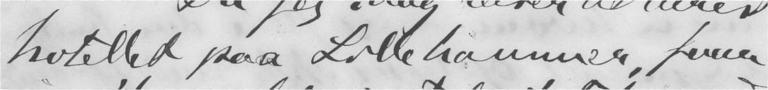hotellet paa Lillehammer, faar
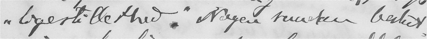"ligestillethed". Nogen saadan beslut-
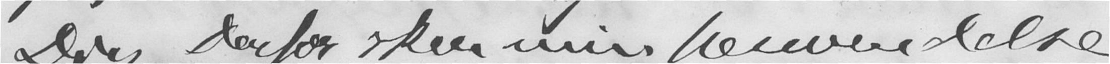Dig. Derfor sker min henvendelse
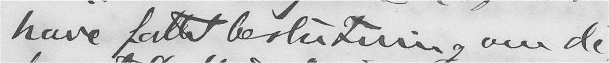have fattet beslutning om de
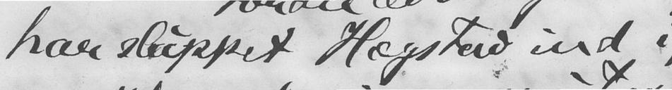har sluppet Hægstad ind i
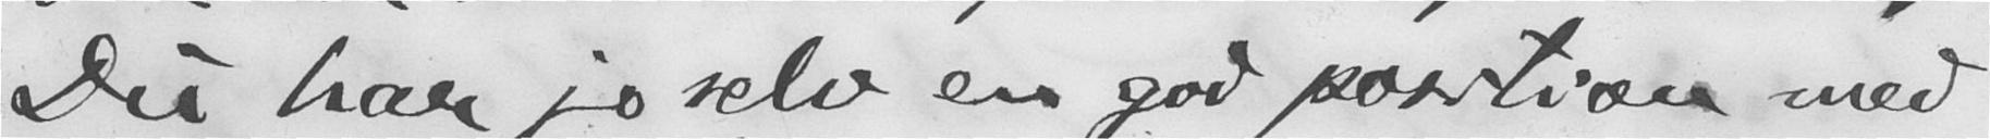Du har jo selv en god position med
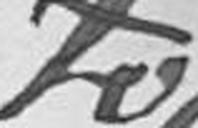to
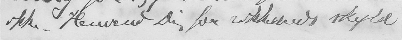ikke. Henvend Dig for sikkerheds skyld
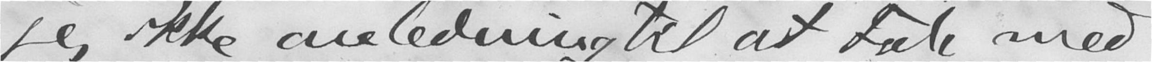jeg ikke anledning til at tale med
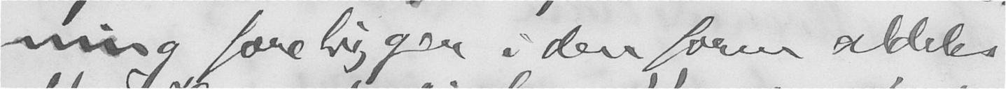ning foreligger i den form aldeles
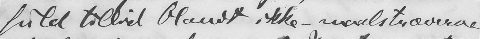fuld tillid blandt ikke-maalstræverne
